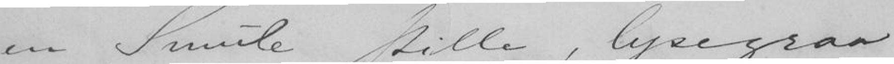en Smule stille, lysegraa
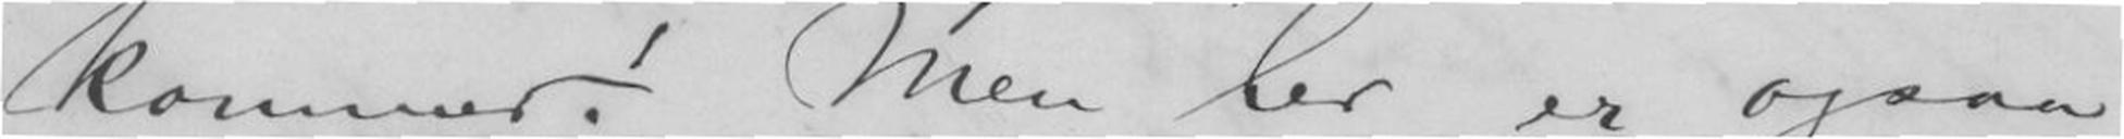kommer! Men her er ogsaa
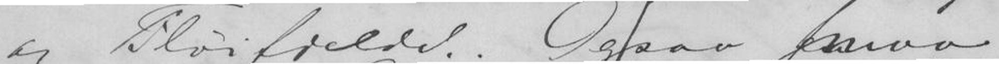og Fløifjeldet. Og saa smaa
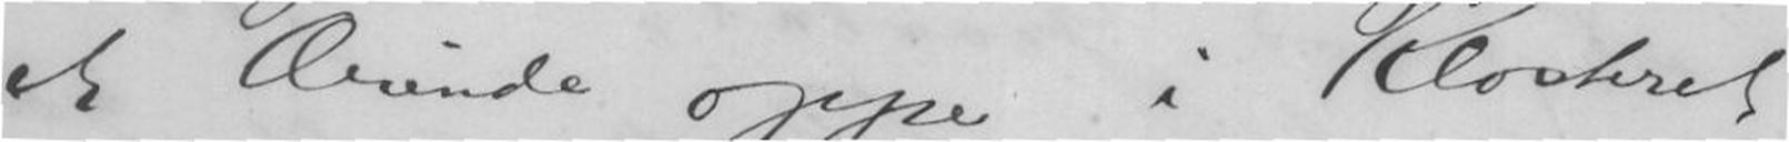et Ærinde oppe i Klosteret,
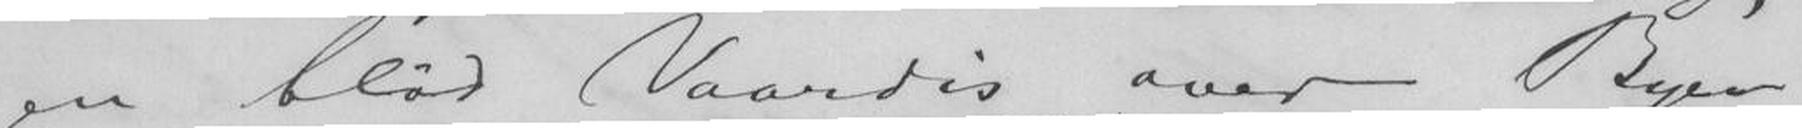en blød Vaardis over Byen
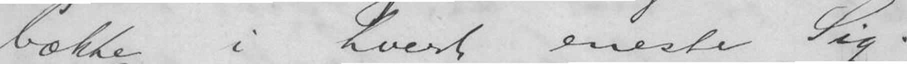bække i hvert eneste Sig.
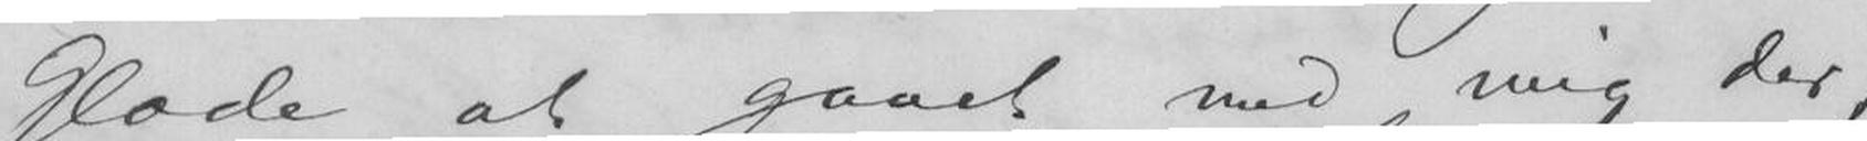Glæde at gaaet med mig der,
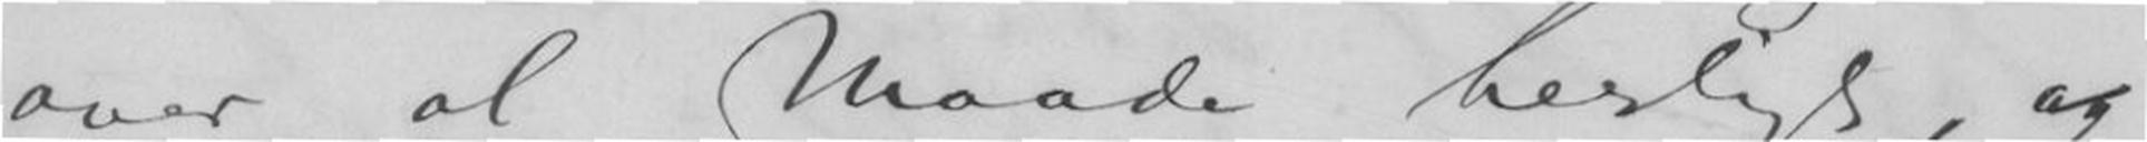over al Maade herligt, og
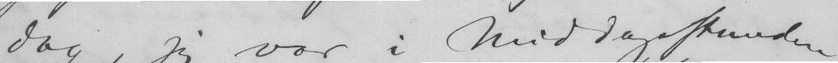dag, jeg var i Middagsstunden
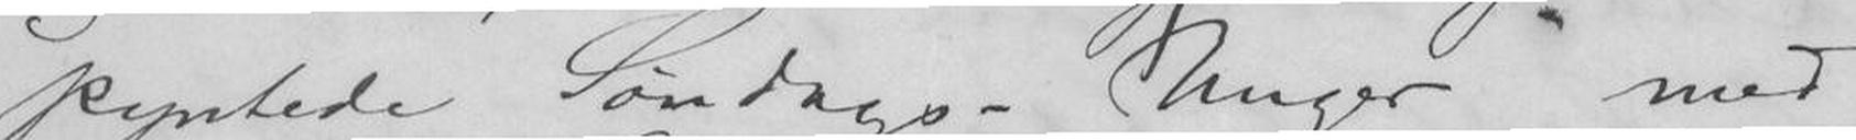pyntede Søndags- Unger med
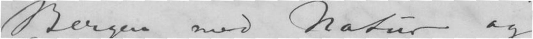Bergen med Natur og
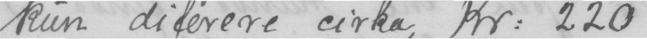kun diferere cirka Kr. 220
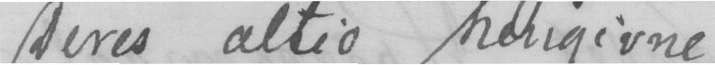Deres altid hengivne
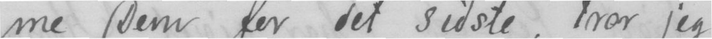me Dem for det sidste , tror jeg
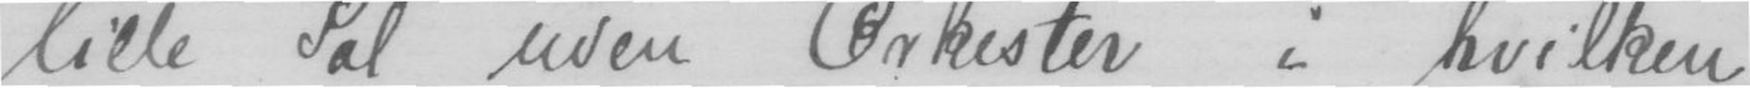lille Sal uden Orkester i hvilken
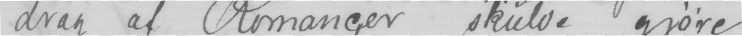drag af Romancer skulde gjøre
